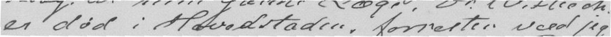er død i Hovedstaden, forresten veed jeg
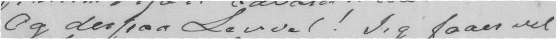Og derpaa Levvel! Jeg faaer vel
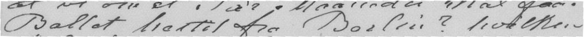Ballet hertil fra Berlin? hvilken
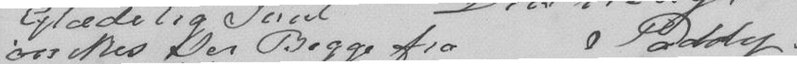ønskes Jer Begge fra Paddy.
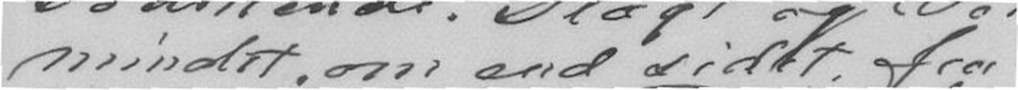mindst om end sidste fra
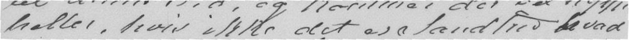heller, hvis ikke det er Sandhed hvad
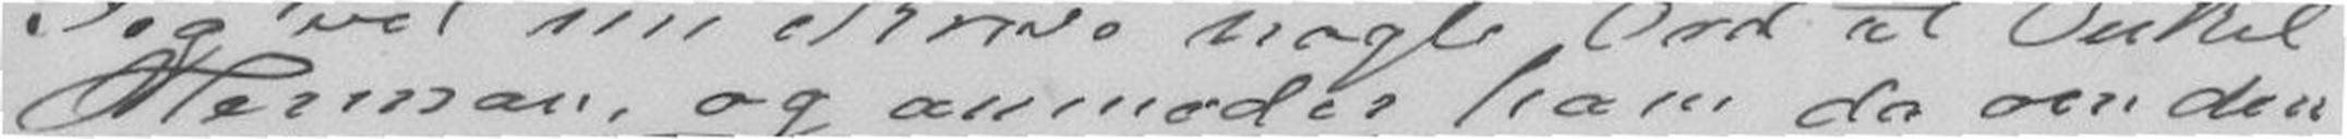Herman, og anmoder ham da om den
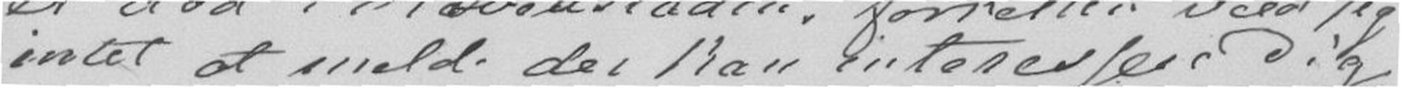intet at melde der kan interessere Dig.
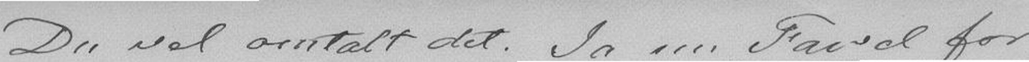Du vel omtalt det. Ja nu Farvel for
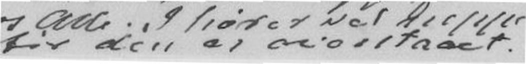før den er overstaaet.
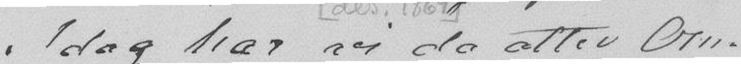Idag har vi atter Om-
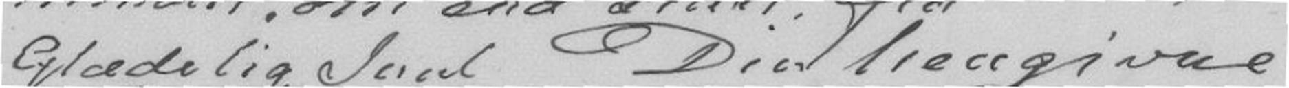Glædelig JuulDin hengivne
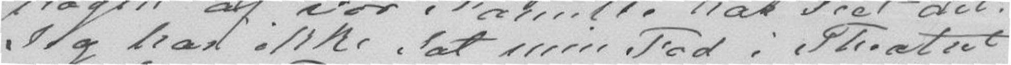Jeg har ikke Sat min Fod i Theatret
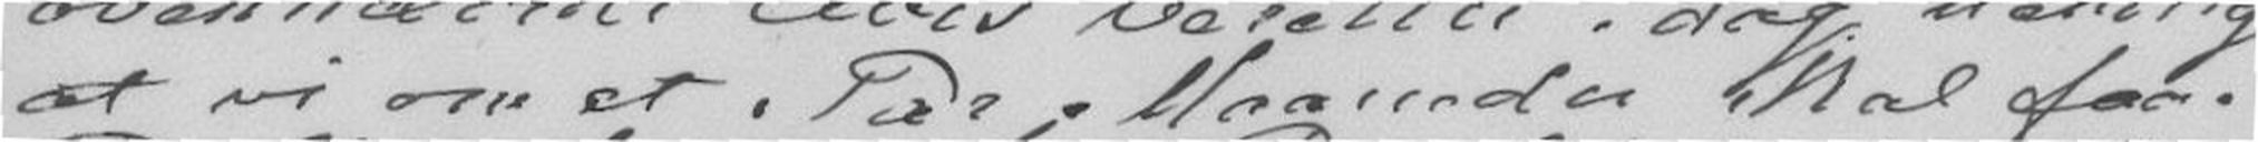at vi om et Par Maaneder skal faae
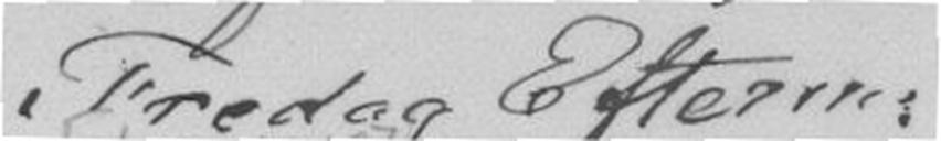Fredag Efterm:
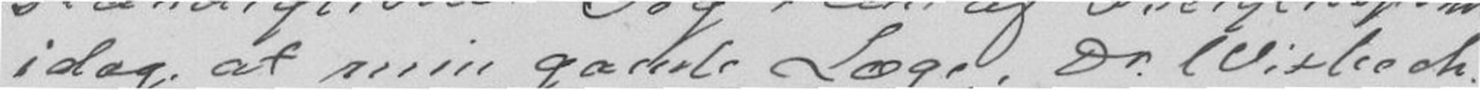idag at min gamle Læge, Dr. Wisbech
